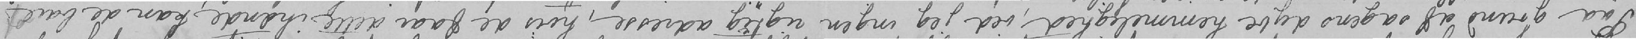Paa grund af sagens dybe hemmelighed, ved jeg ingen riktig adresse, hvis de faar dette ihænde, kan de bare
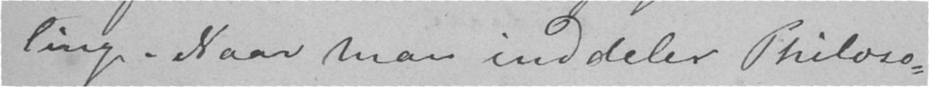ling. Naar man inddeler Philoso-
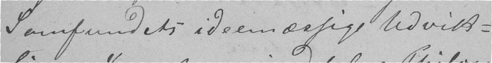Samfundets ideemæssige Udvik-
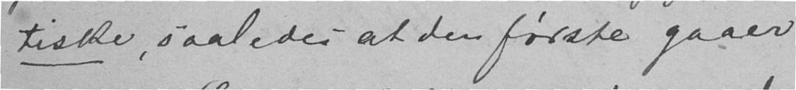tiske, saaledes at den første gaaer
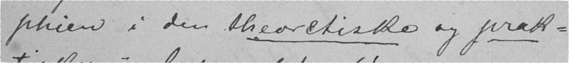phien i den theoretiske og prak-
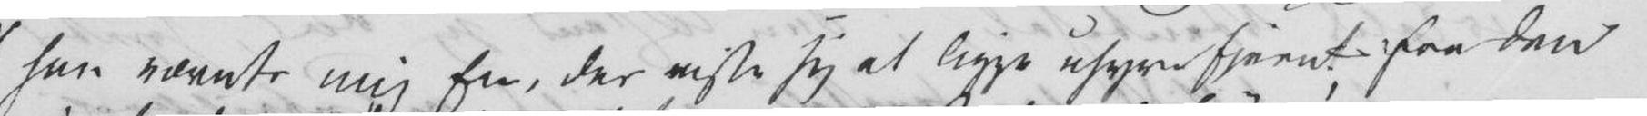han nævnte mig En, der viste sig at ligge uhyre fjernt fra den
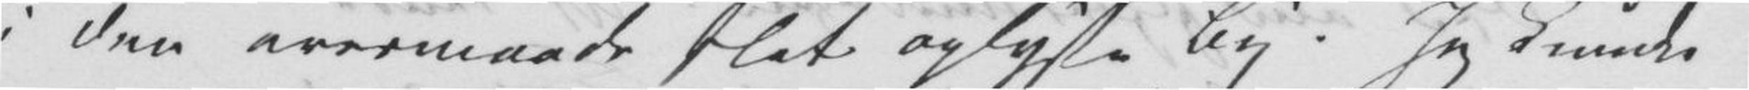i den overmaade slet oplyste By. Jeg kunde
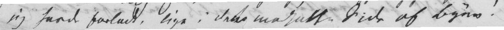jeg havde forladt, lige i den modsatte Side af Byen!
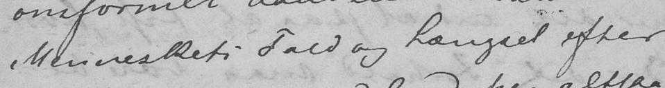Menneskets Fald og Længsel efter
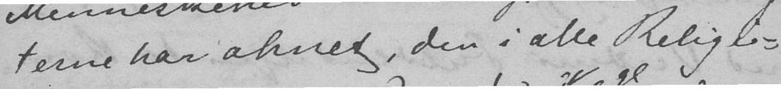terne har ahnet, den i alle Relig-
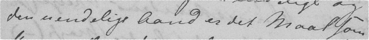den uendelige Aand er det Maal som
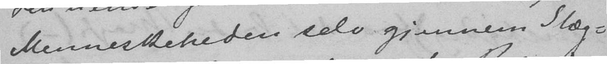Menneskeheden selv gjennem Slæg-
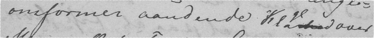onsformer aandende Klage over
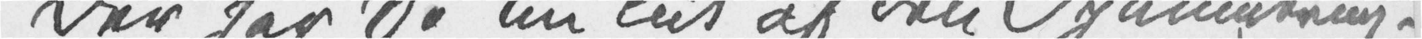Der har De nu lidt af den Opmuntring,
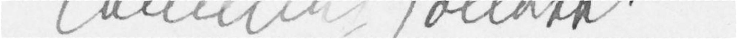Camilla Collett.
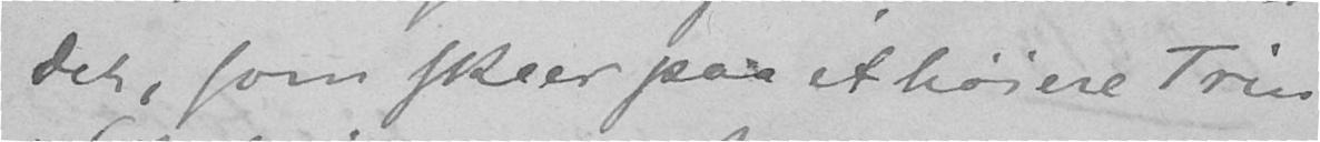det, som skeer paa et høiere Trin
